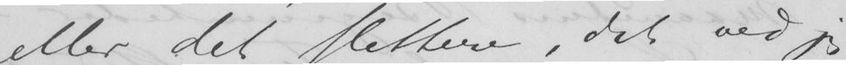eller det slettere, det ved jeg
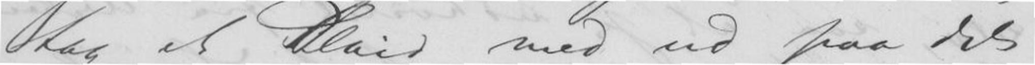tog et Plaid med ud paa det
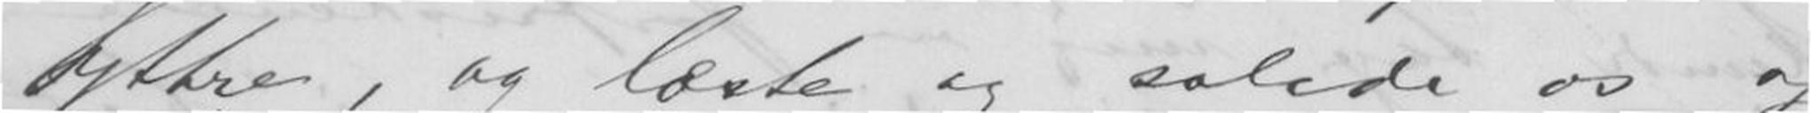yttre, og læste og solede os og
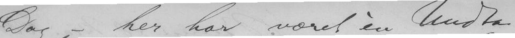Dog - her har været én Undta-
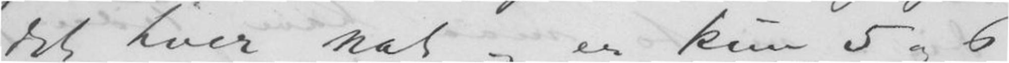det hver Nat og er kun 5 og 6
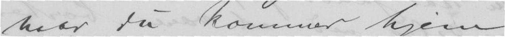naar Du kommer hjem,
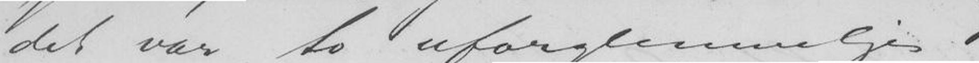det var to uforglemmelige
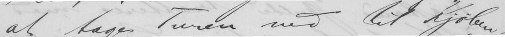at tage Turen ned til Kjøben-
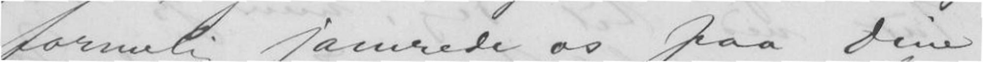formelig jamrede os paa Dine
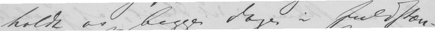holdt os begge Dage i fuldstæn-
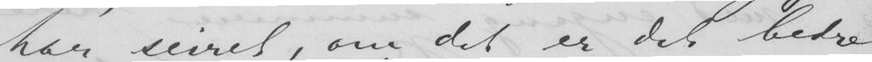har seiret, om det er det bedre
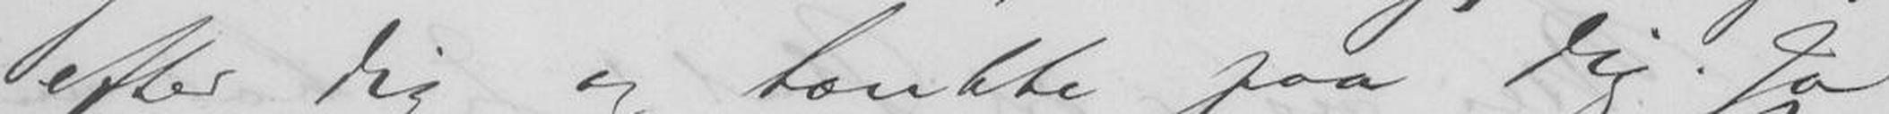efter Dig og tænkte paa Dig. Ja
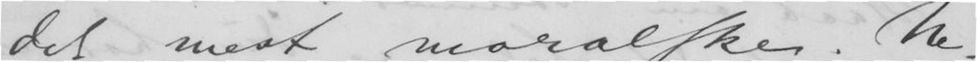det mest moralske. Ne-
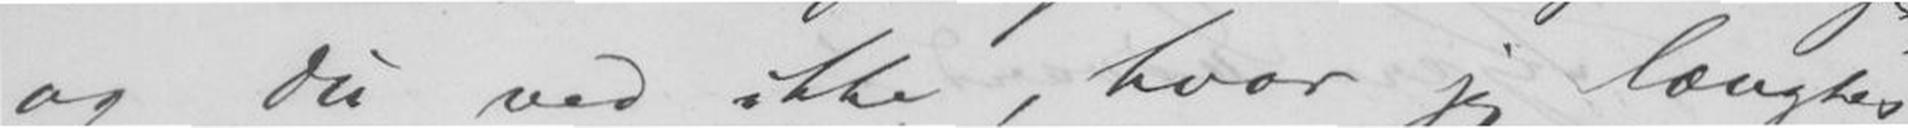og Du ved ikke, hvor jeg længtes
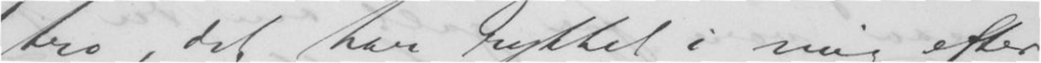tro, det har rykket i mig efter
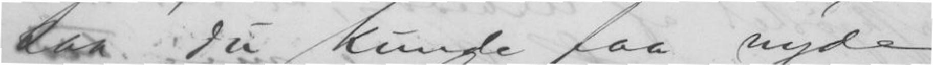saa Du kunde faa nyde
